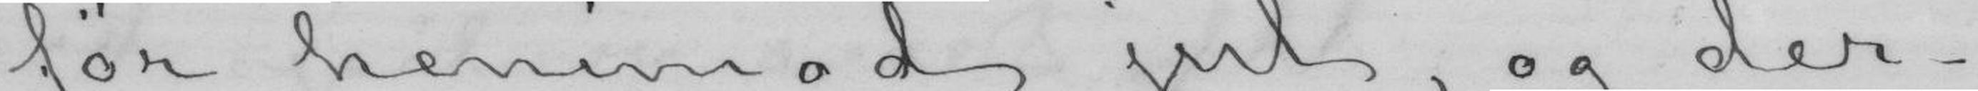før henimod jul, og der-
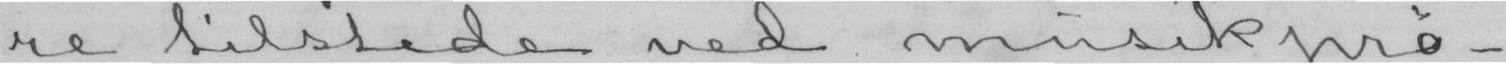re tilstede ved musikprø-
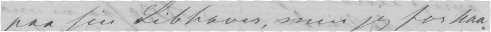paa sin Libhaver, men jeg for haa-
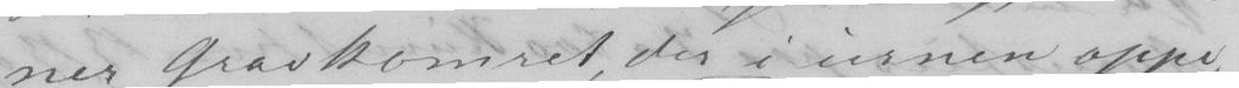ner Gravkamret, der i urnen oppe-
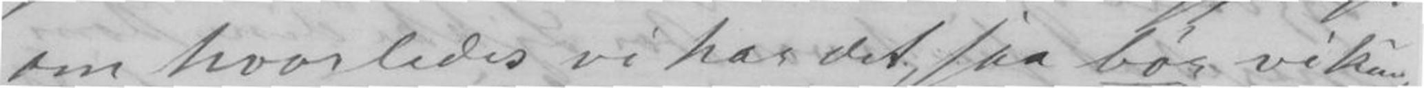om hvorledes vi har det saa bør vi kun-
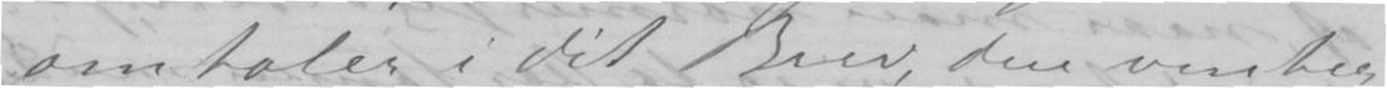omtaler i dit Brev, den venter
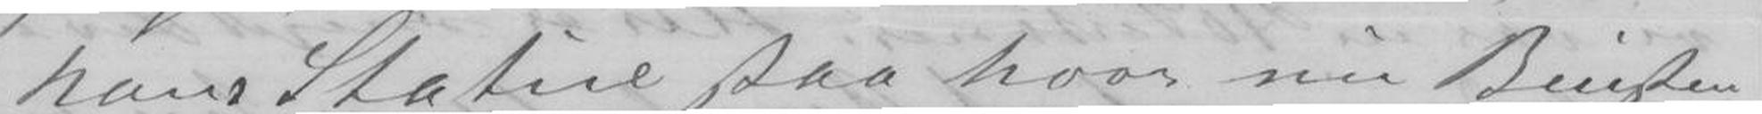hans Statue saa hvor nu Buesten
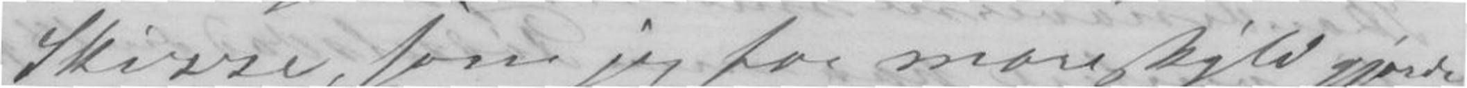Skizze, som jeg for moroskyld gjorde
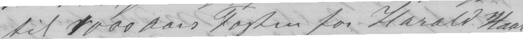til 1000 Aars Fæsten for Harald Haar-
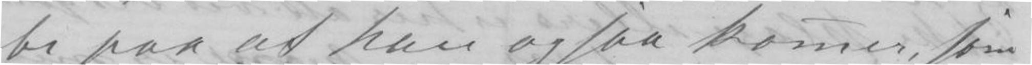be paa at han ogsaa komer, som
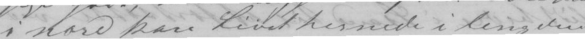i nord kan Livet hernede i lengden
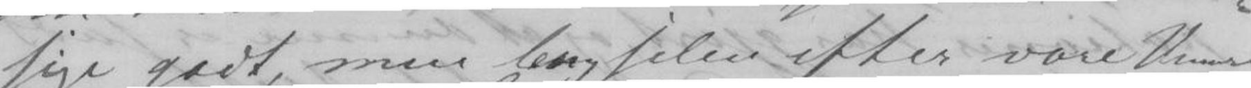ne sige godt, men lengselen efter vore Venner
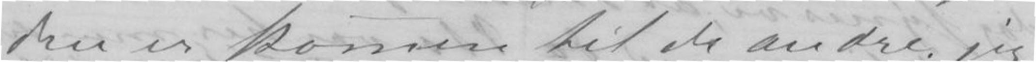den er komen til de andre; jeg
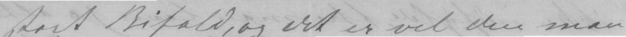stort Bifald, og det er vel den man
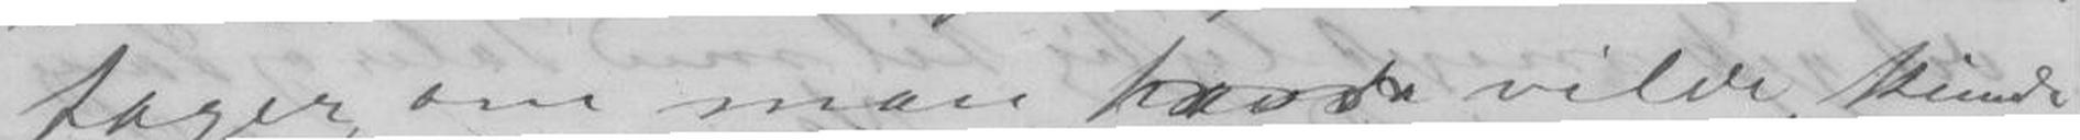fager, om man vilde, kunde
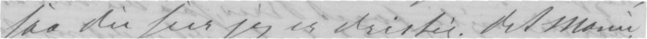saa du seer jeg er dristig. Det Monu-
